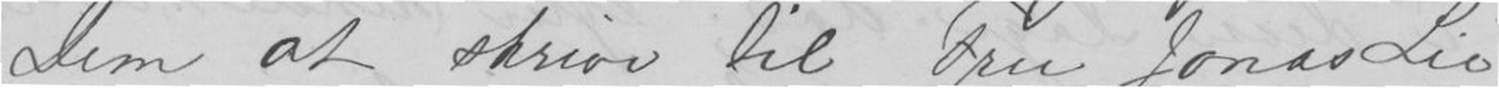Dem at skrive til Fru Jonas Lie
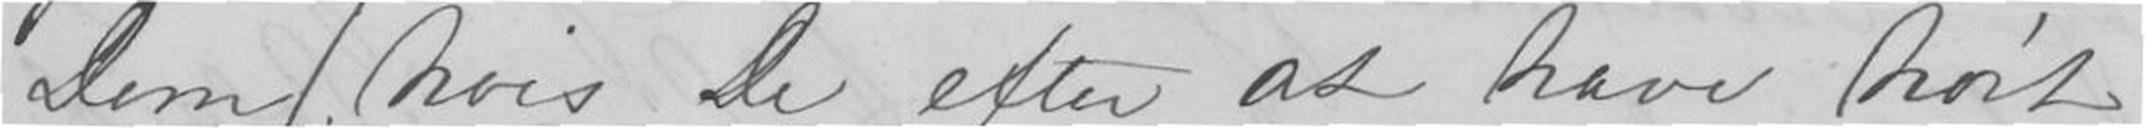Dem, (hvis De efter at have hørt
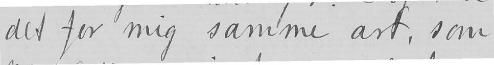det for mig samme art, som
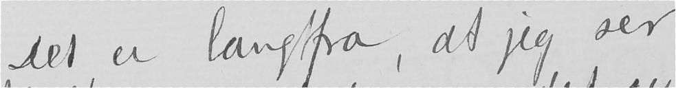Det er langtfra, at jeg ser
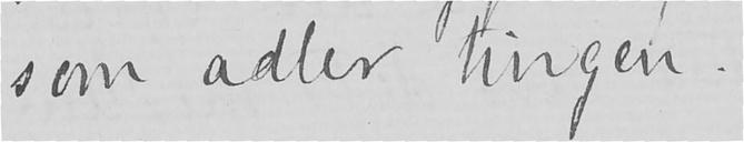som adler tingen.
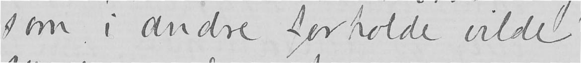som i andre forholde vilde
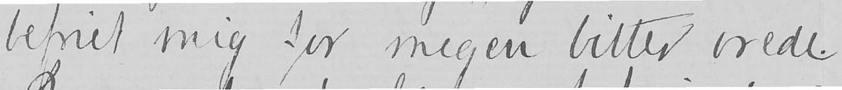befriet mig for megen bitter vrede.
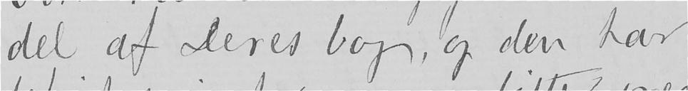del af Deres bog, og den har
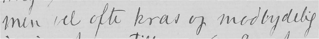men vel ofte kras og modbydelig
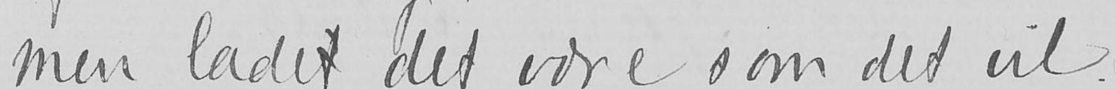men ladet det være, som det vil.
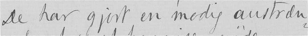De har gjort en modig anstræn-
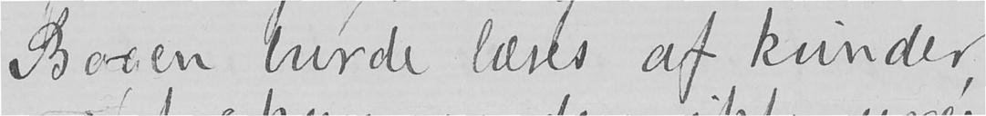Bogen burde læses af kvinder,
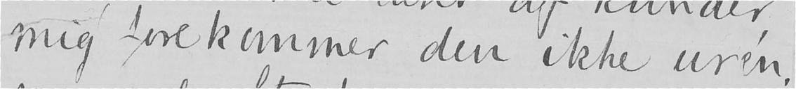mig forekommer den ikke uren,
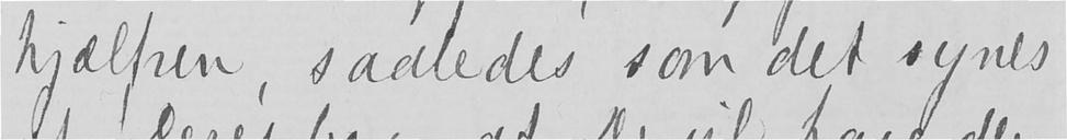hjælpen, saaledes som det synes
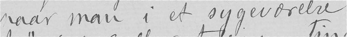naar man i et sygeværelse
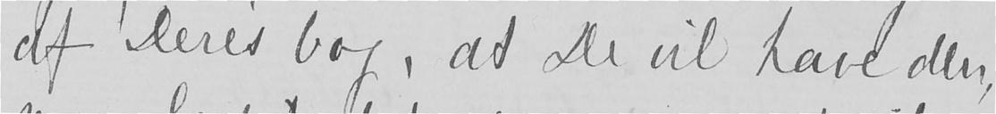af Deres bog, at De vil have den,
